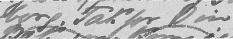borg.Tak for Din
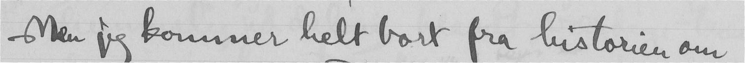Men jeg kommer helt bort fra historien om
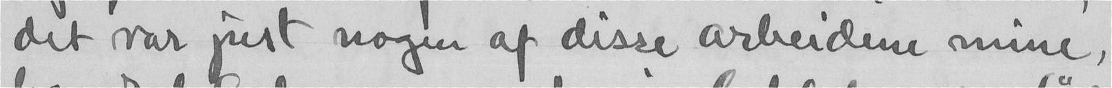det var just nogen af disse arbeidene mine,
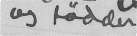og fødder
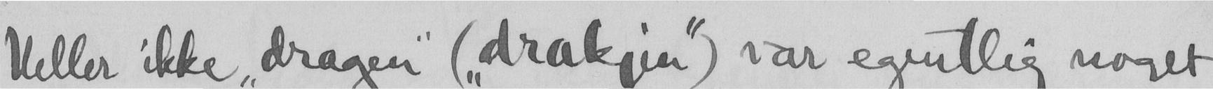Heller ikke "dragen" ("drakjen") var egentlig noget
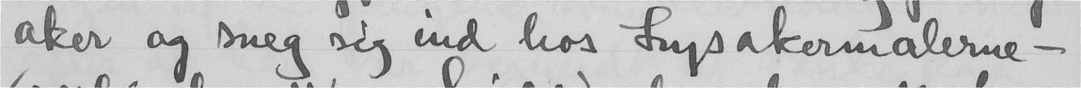aker og snig sig ind hos Lysakermalerne -
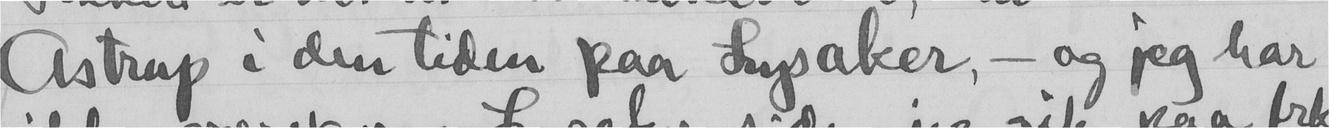Astrup i den tiden paa Lysaker, - og jeg har
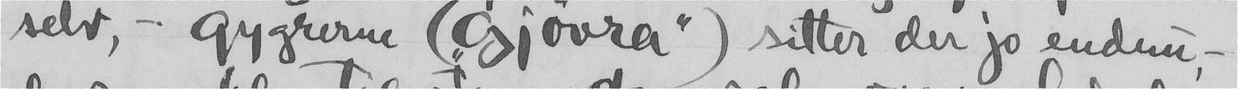selv, - gygrerne ("gjøvra") sitter der jo endnu, -
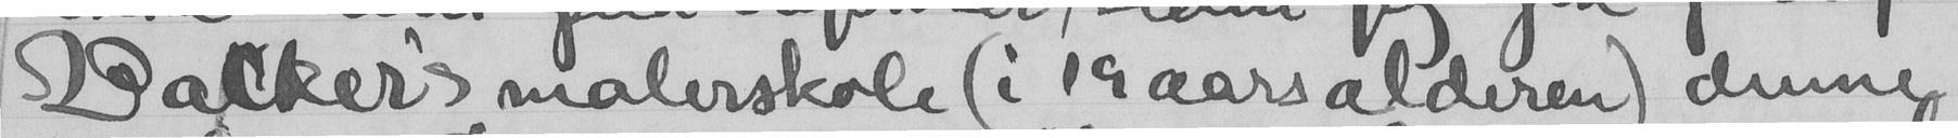Backers malerskole (i 19 aars alderen) denne
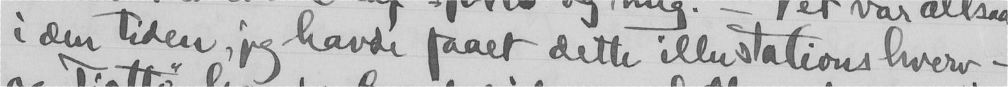i den tiden, jeg havde faaet dette illustations hverv
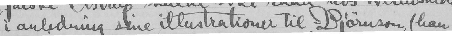i anledning sine illustrationer til Bjørnson, (han
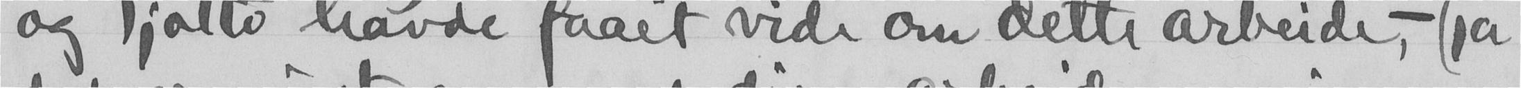og Tjøttø havde faaet vide om dette arbeide, - (ja
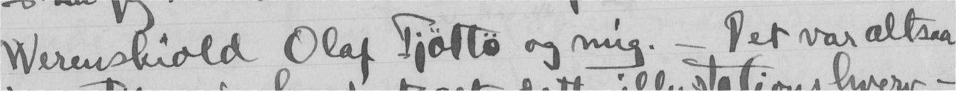Werenskiold Olaf Tjøttø og mig. - Det var altsaa
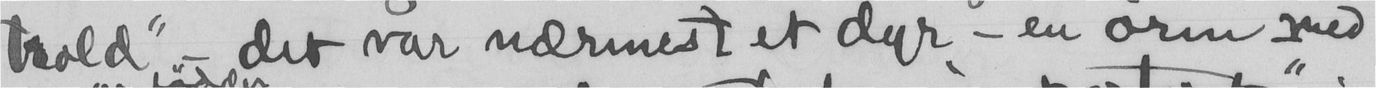"trold", - det var nærmest et dyr - en orm med
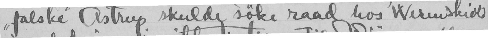"falske" Astrup skulde søke raad hos Werenskiold
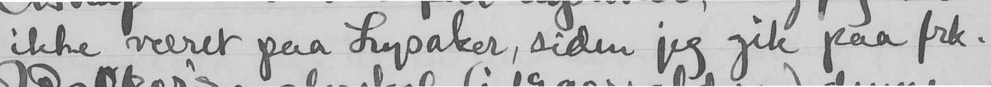ikke været paa Lysaker, siden jeg gik paa frk.
